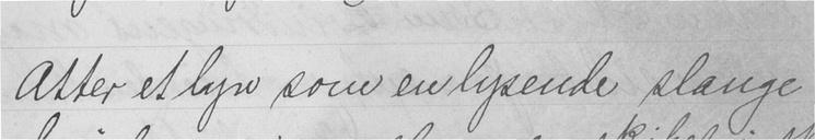Atter et lyn som en lysende slange
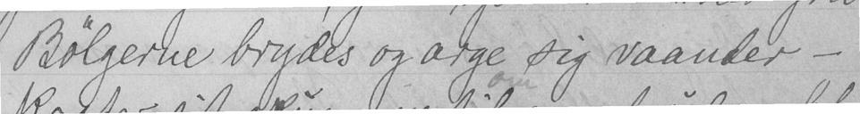Bølgerne brydes og arge sig vaander -
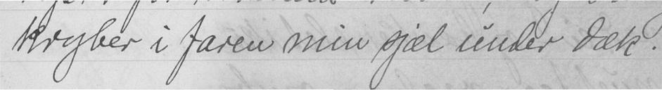kryber i faren min sjæl under dæk.
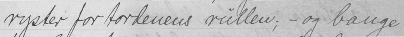ryster for tordenens rullen; - og bange
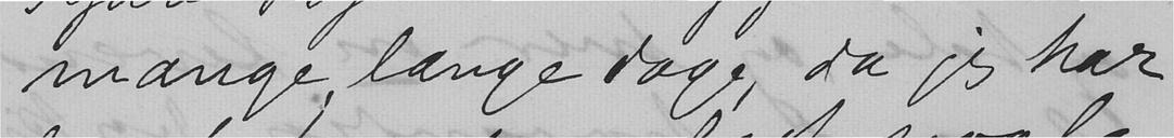mange lange dage, da jeg har
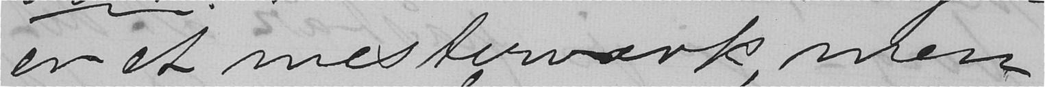er et mesterværk, men
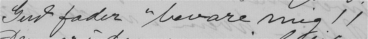Gud fader bevare mig!!
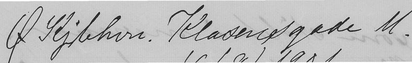Ø Kjbhvn. Klasensgade 11.
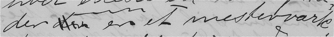der der er et mesterværk.
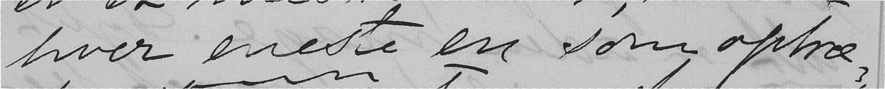hver eneste en som optræ-
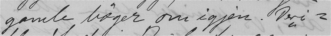gamle bøger om igjen. Deri-
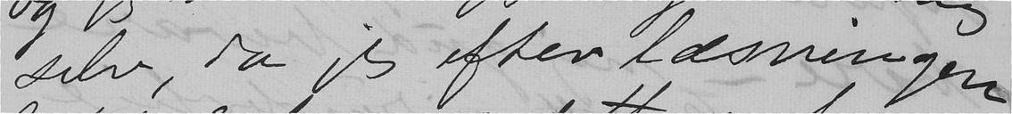selv da jeg efter læsningen
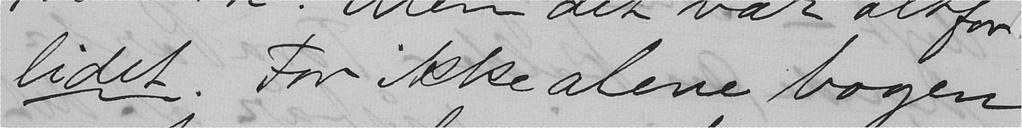lidet. For ikke alene bogen
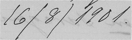16/8/1901.
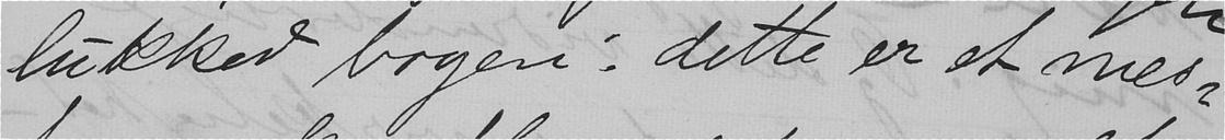lukked bogen: dette er et mes-
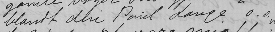blandt den "Paul Lange" o.s.v.
